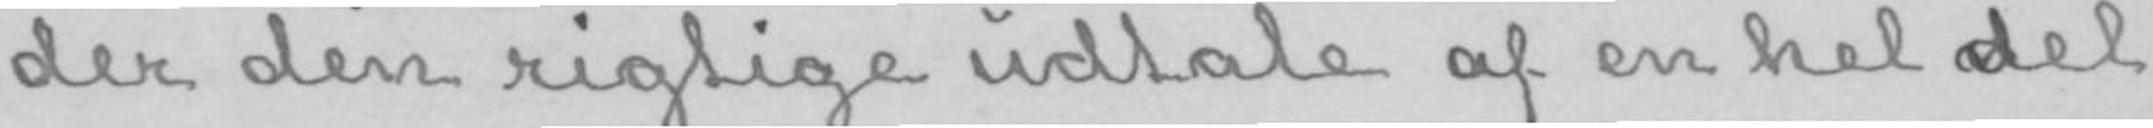der den rigtige udtale af en hel del
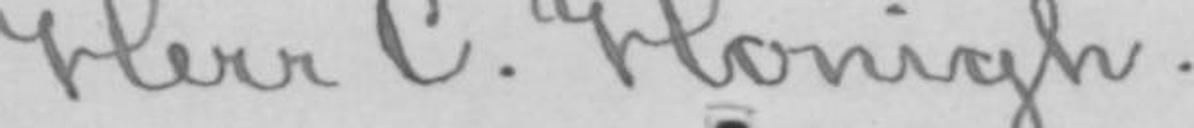Herr C. Honigh.
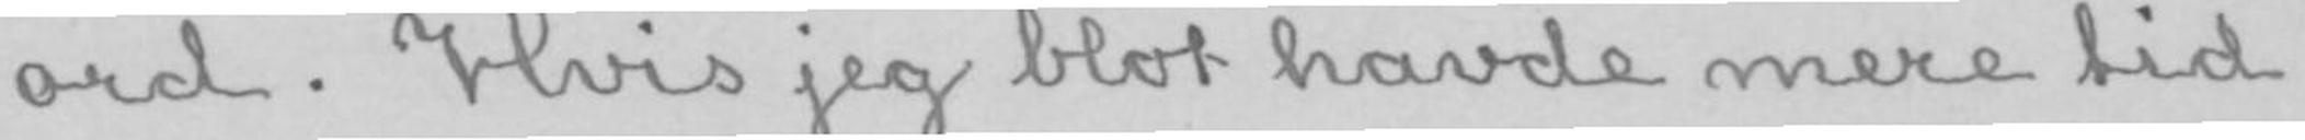ord. Hvis jeg blot havde mere tid
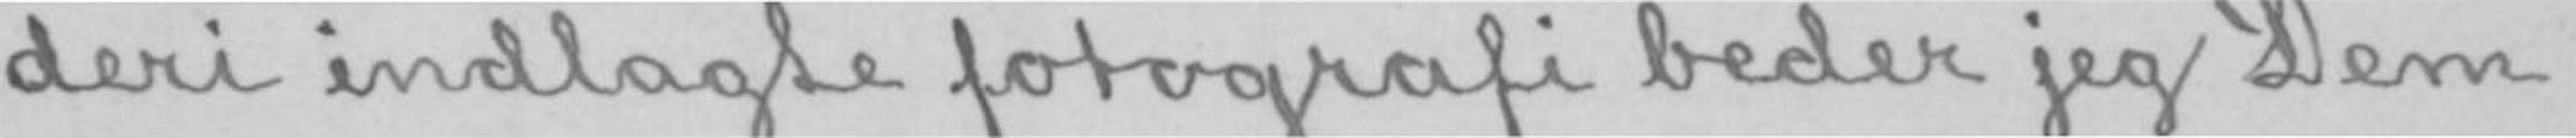deri indlagte fotografi beder jeg Dem
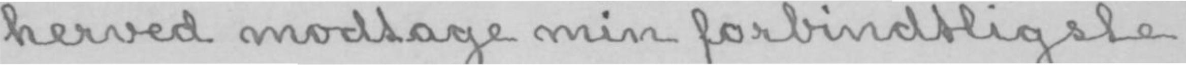herved modtage min forbindtligste
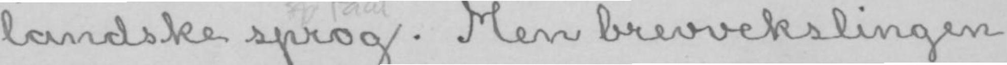landske sprog. Men brevvekslingen
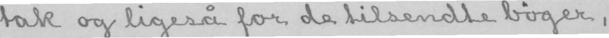tak og ligeså for de tilsendte bøger,
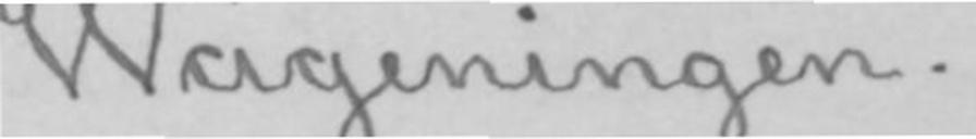Wageningen.
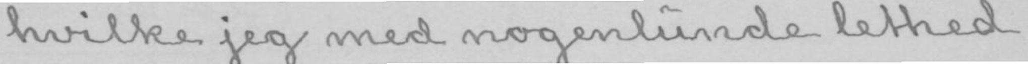hvilke jeg med nogenlunde lethed
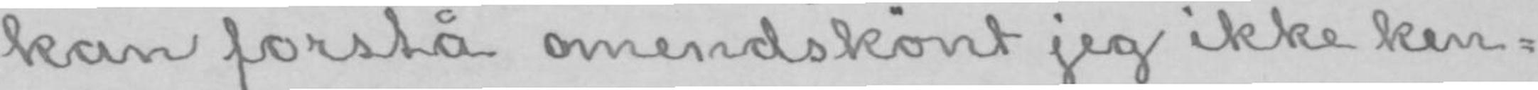kan forstå omendskønt jeg ikke ken-
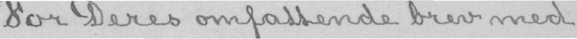For Deres omfattende brev med
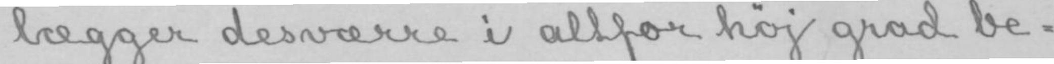lægger desværre i altfor høj grad be-
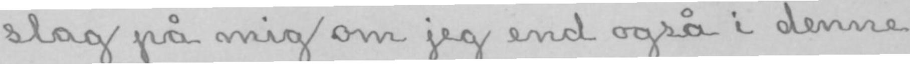slag på mig om jeg end også i denne
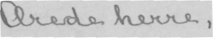Ærede herre,
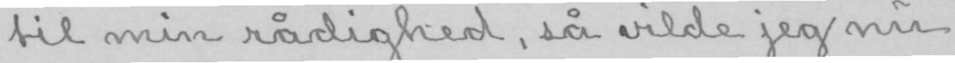til min rådighed, så vilde jeg nu
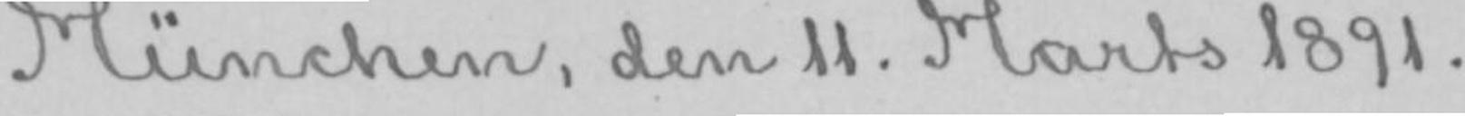München, den 11. Marts 1891.
